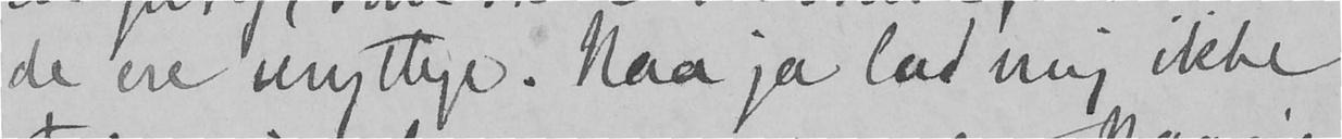de ere unyttige. Naa ja lad mig ikke
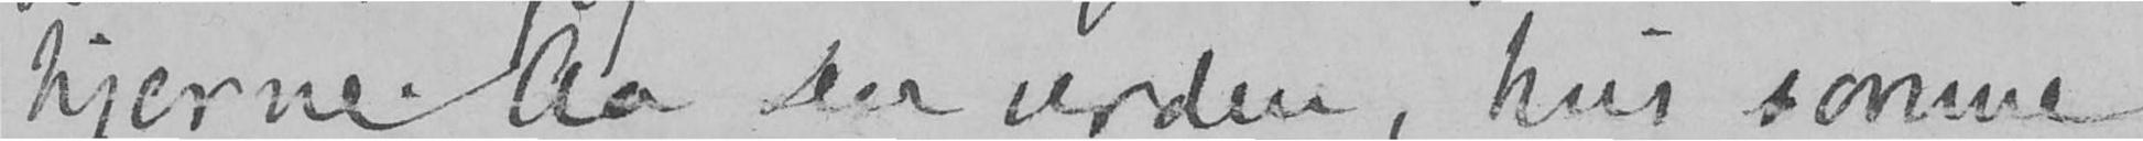hjerne. Aa Du verden, hvis somme
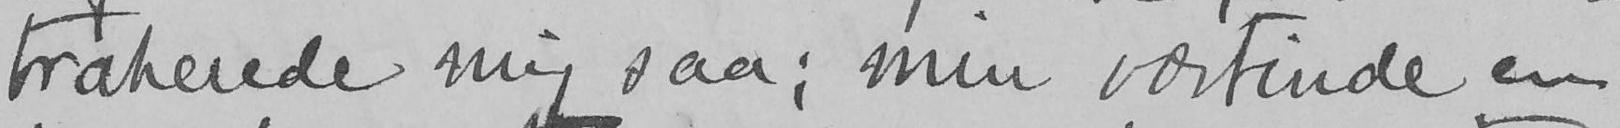traherede mig saa: min værtinde en
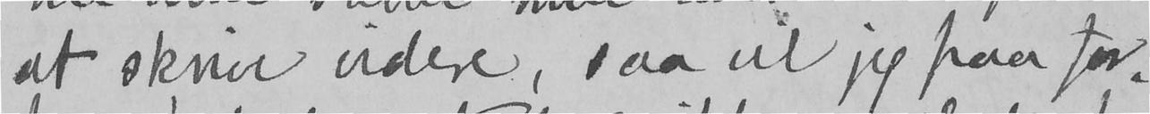at skrive videre, saa vil jeg paa for-
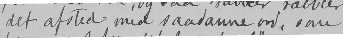det afsted med saadanne ord, som
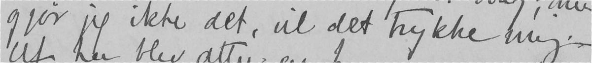gjør jeg ikke det, vil det trykke mig.
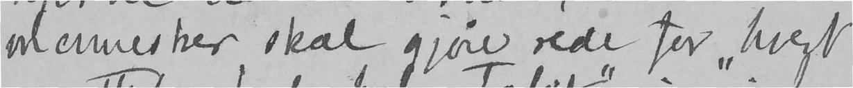mennesker skal gjøre rede for "hvert
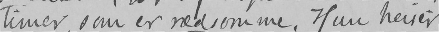timer, som er rædsomme. Hun heiser
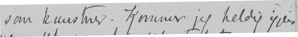som kunstner. Kommer jeg heldig igjen-
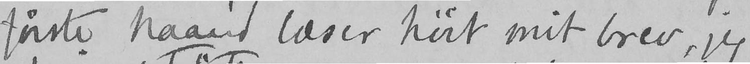første haand læser høit mit brev, jeg
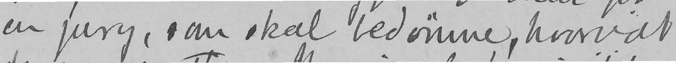en jury, som skal bedømme, hvorvidt
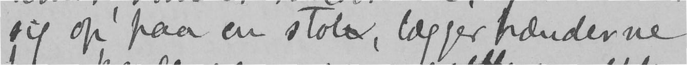sig op paa en stole, lægger hænderne
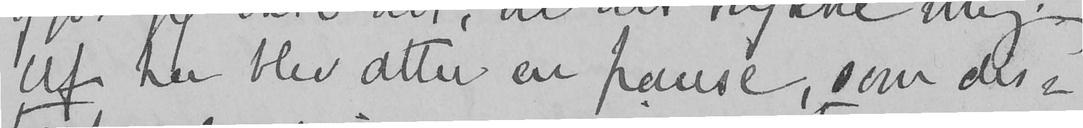Uf her blev atter en pause, som dis-
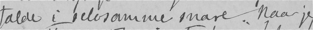falde i selvsomme snare. Naar jeg
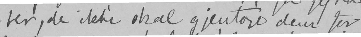ber, de ikke skal gjentage dem for
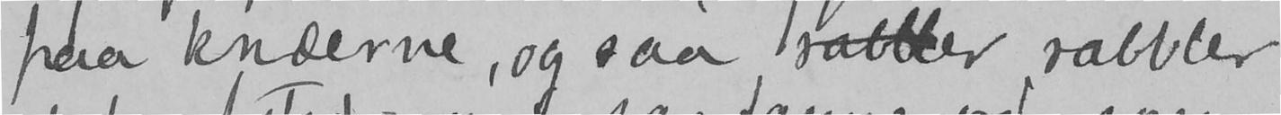paa knæerne, og saa rabbler rabbler
